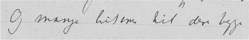Og mange hilsener til dere begge
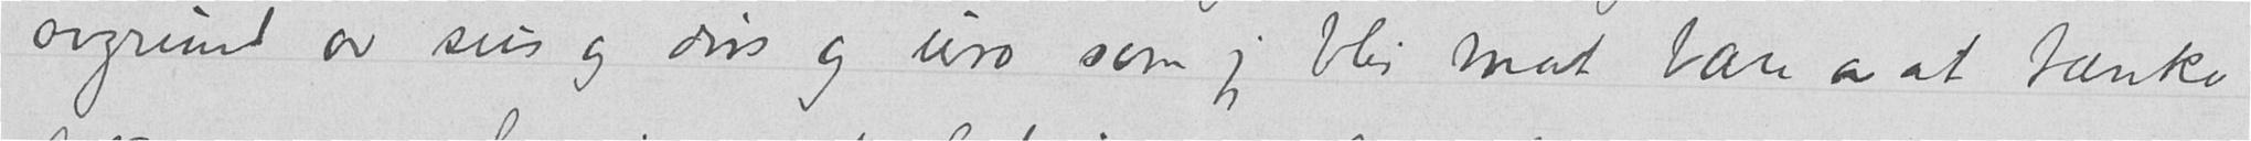avgrund av sus og dus og uro som jeg blir mat bare av at tænke
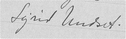Sigrid Undset.
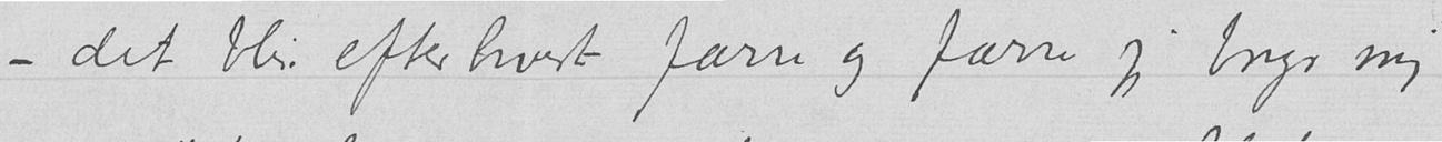– det blir efterhvert færre og færre jeg bryr mig
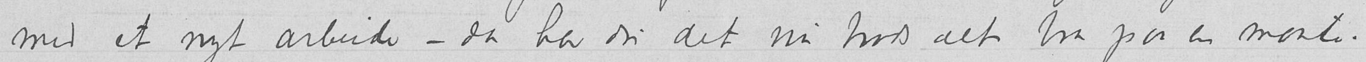med et nyt arbeide – da har du det nu trods alt bra paa en maate.
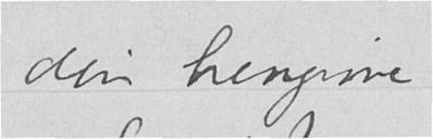din hengivne
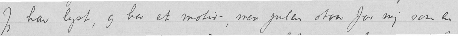Jeg har lyst, og har et motiv –, men julen staar for mig som en
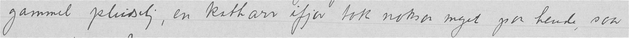gammel pludselig, en katharr ifjor tok noksaa meget paa hende, saa
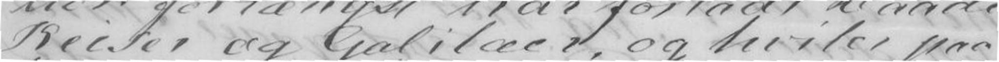Keiser og Galilæer, og hviler paa
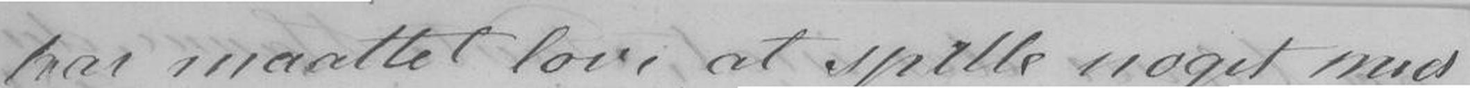har maattet love at spille noget med
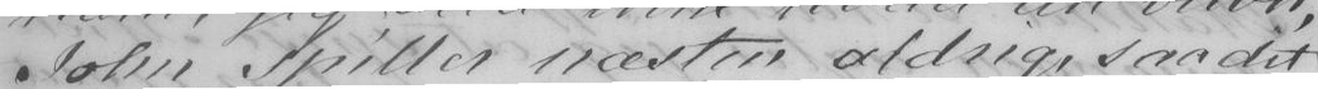John spiller næsten aldrig saa det
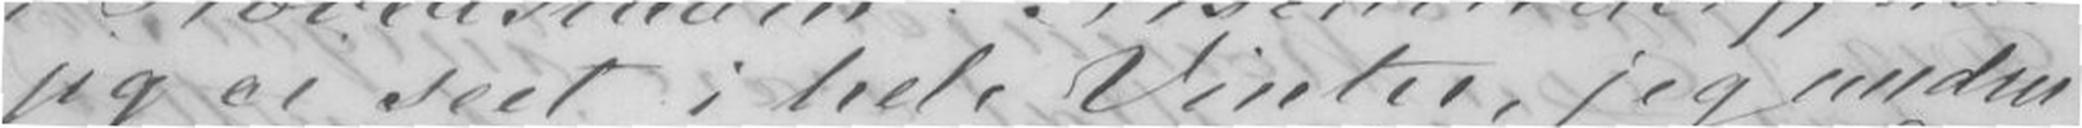jeg ei seet i hele Vinter, jeg undrer
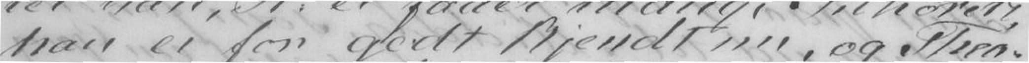han er for godt kjendt nu, og Thea-
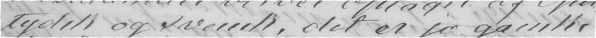tydsk og svensk, det er jo ganske
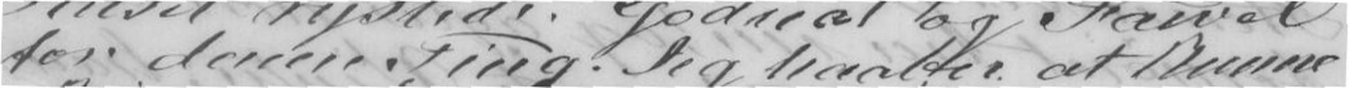for denne Ting. Jeg haaber at kunne
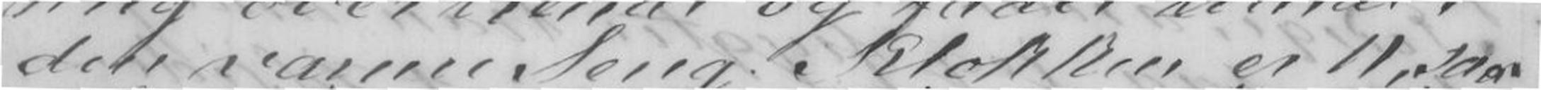den varme Seng. Klokken er 11, saa
